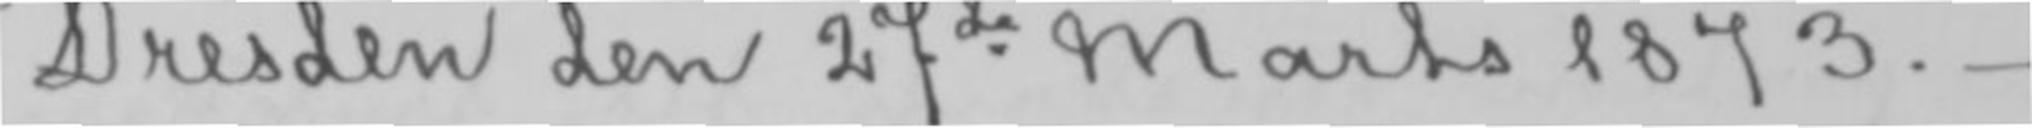Dresden den 27de Marts 1873.-
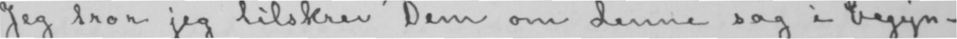Jeg tror jeg tilskrev Dem om denne sag i begyn-
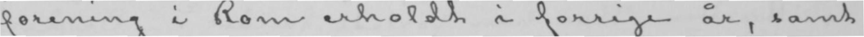forening i Rom erholdt i forrige år, samt
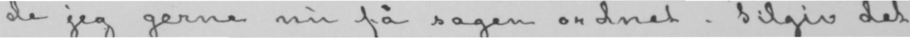de jeg gerne nu få sagen ordnet. Tilgiv det
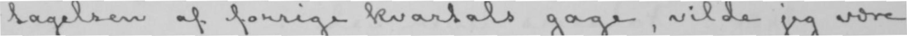tagelsen af forrige kvartals gage, vilde jeg være
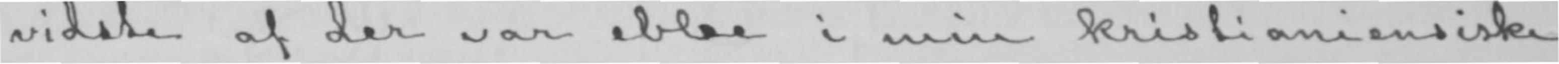vidste af der var ebbe i min kristianiensiske
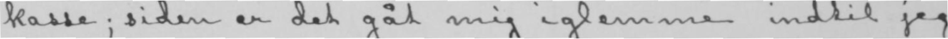kasse; siden er det gåt mig iglemme indtil jeg
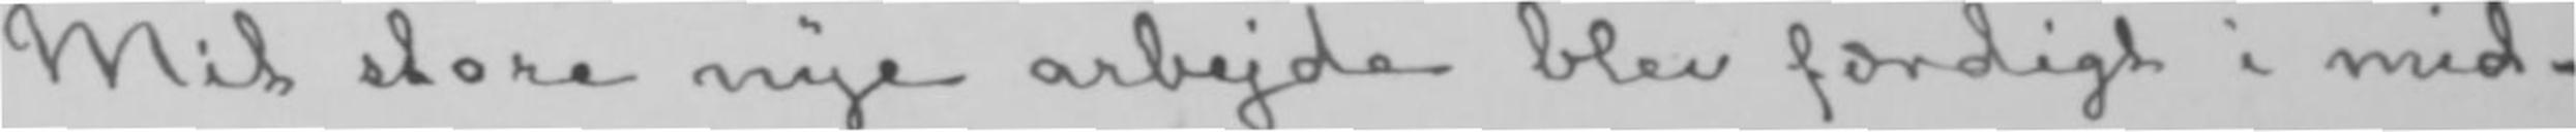Mit store nye arbejde blev færdigt i mid-
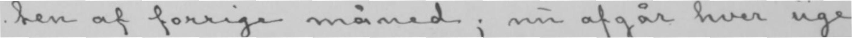ten af forrige måned; nu afgår hver uge
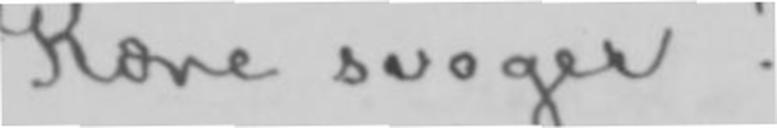Kære svoger!
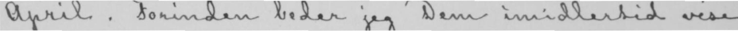April. Forinden beder jeg Dem imidlertid vise
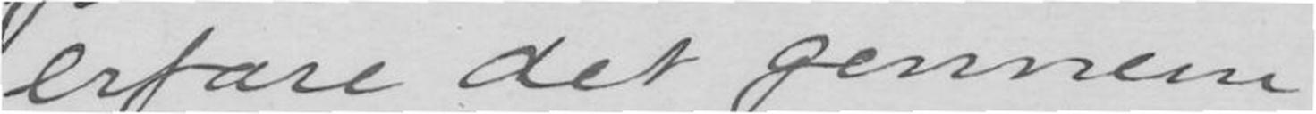erfare det gennem
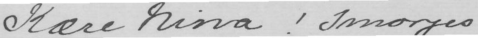Kære Nina! Imorges
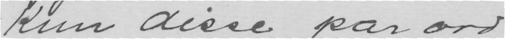Kun disse par ord
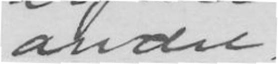andre.
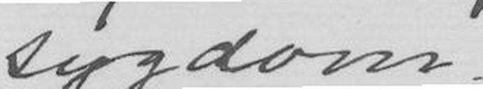sygdom.
